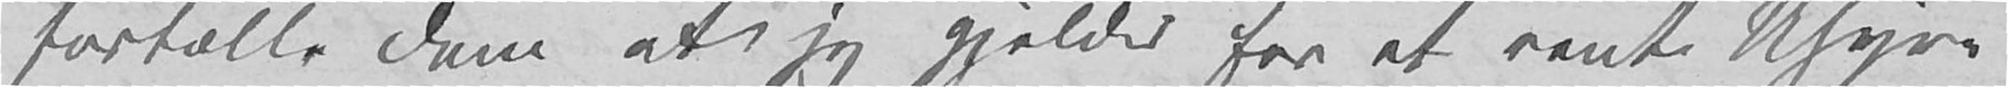fortælle Dem at jeg gjelder for et rent Uhyre
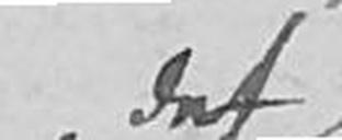det
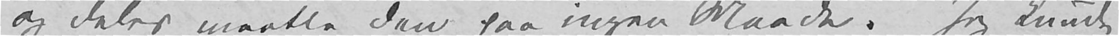og deles maatte den paa ingen Maade. Jeg kunde
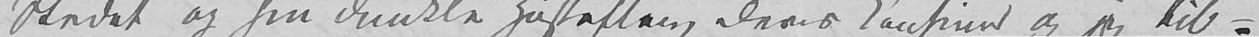Stedet og hin dunkle Høstaften, Deres Cousiner og jeg til¬
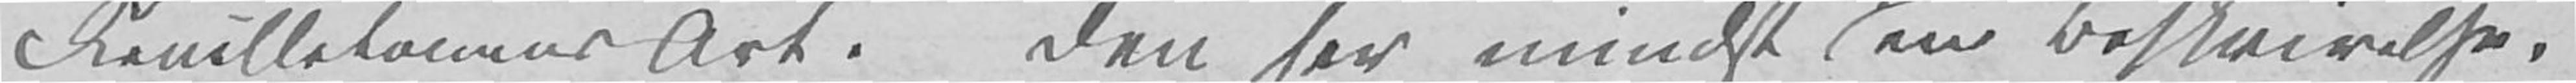Feuilletonens Art. Den har mindst en Beskrivelse.
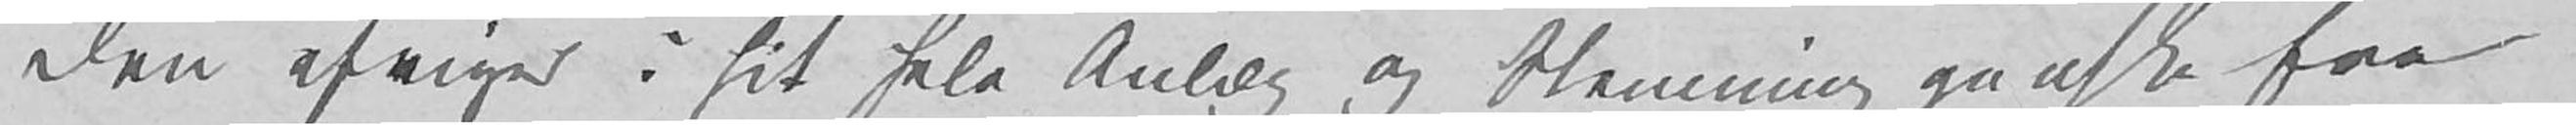Den afviger i sit hele Anlæg og Stemning ganske fra
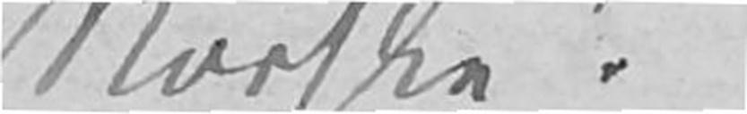Norske!
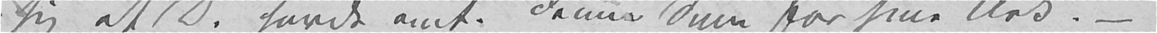sig at D. havde omt. denne Sum for sine Ark. –
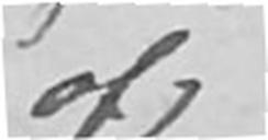af
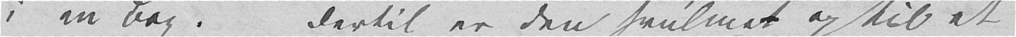i en Bog. Dertil er den svulmet op til et
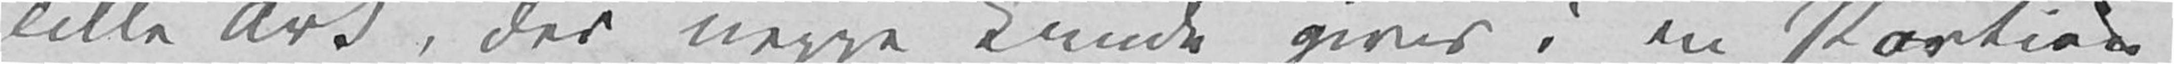lille Ark, der neppe kunde gives i en Portion,
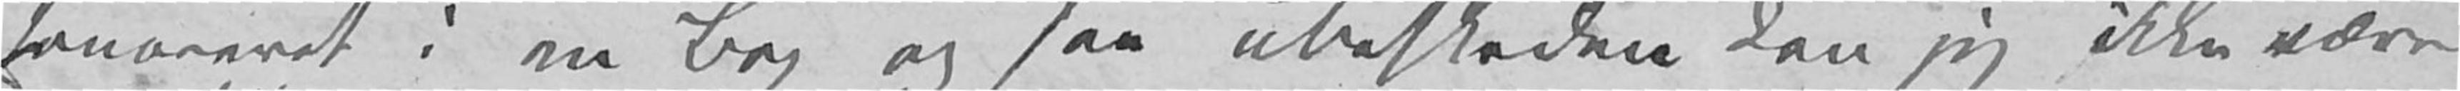honoreret i en Bog og saa ubeskeden kan jeg ikke være
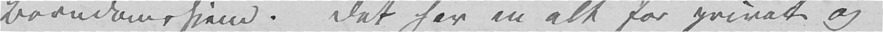Barndomshjem. Det har en alt for privat og
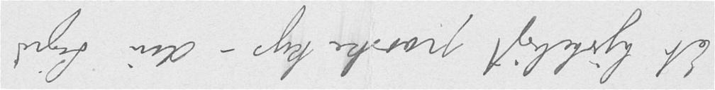Et hjerteligt paaskekys – din Sigrid
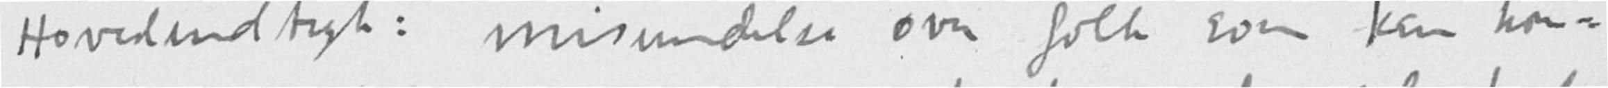Hovedindtryk: misundelse over folk som kan kon-
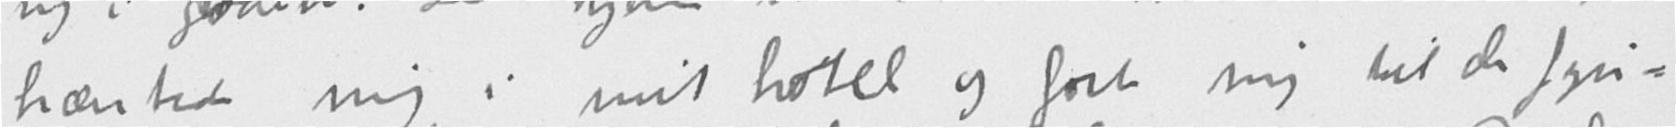hæntede mig i mit hotel og førte mig til de fysi-
